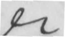er
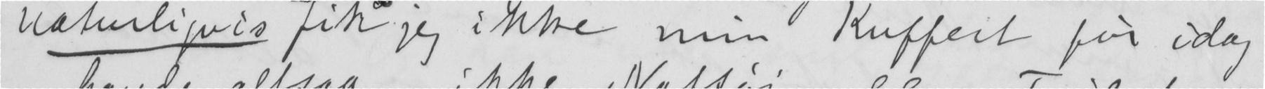naturligvis fik jeg ikke min Kuffert før idag
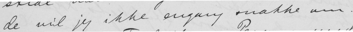de vil jeg ikke engang snakke om.
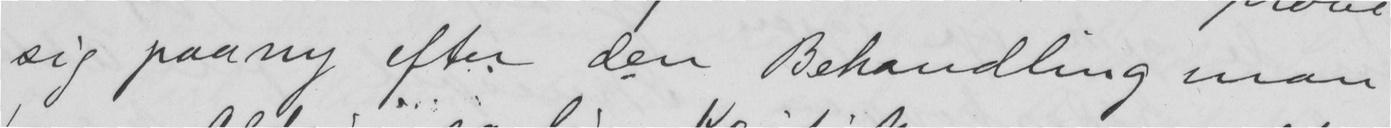sig paany efter den Behandling man
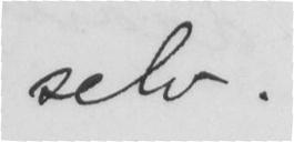selv.
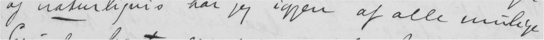og naturligvis har jeg igjen af alle mulige
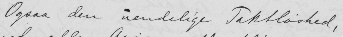Ogsaa den uendelige Taktløshed,
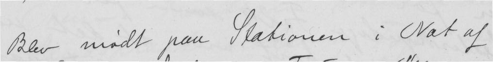Blev mødt paa Stationen i Nat af
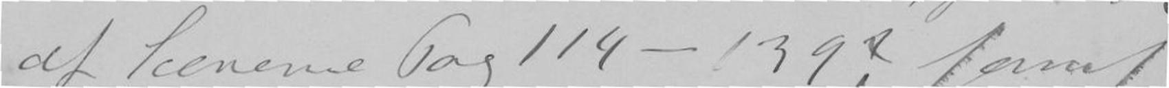af Scenene Pag 114 - 139? Samt
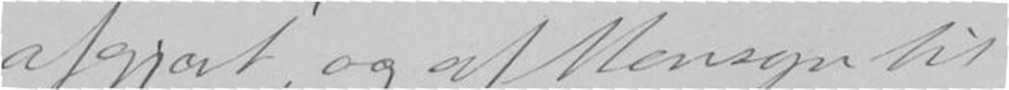og afgjort, og af Hensyn til
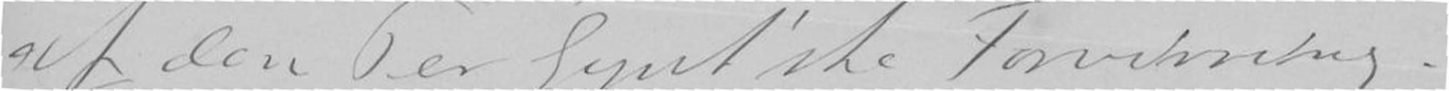af den Per Gynt`ske Forvirring.
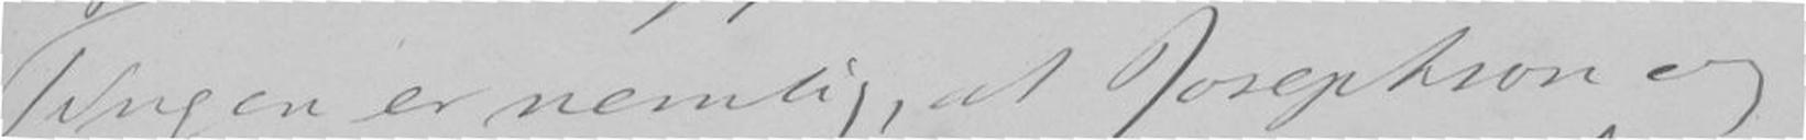Tingen er nemlig, at Josephson og
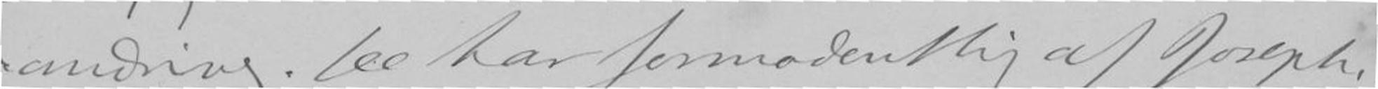=andring. De har formodentlig af Joseph-
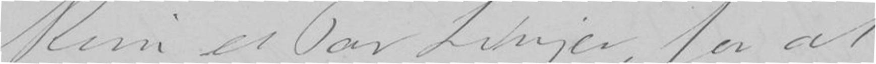Kun et Par Linjer, for at
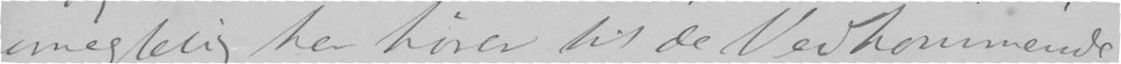unegtelig her hører til de Vedkommende,
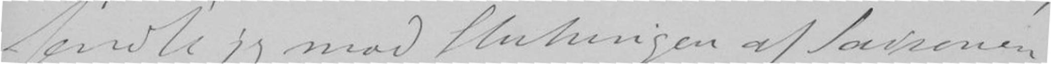sendte jeg mod Slutningen af Saisonen
